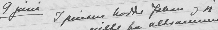9 juni I pinsen hadde Johan og jeg
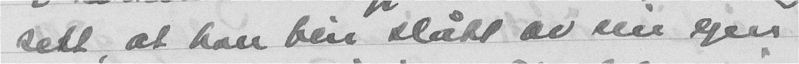sett, at han blir slått av sin egen
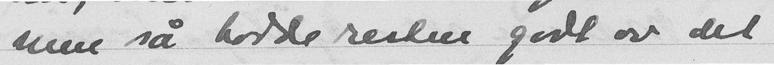men så hadde resten godt av det
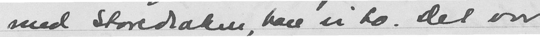med Storedraken, bare vi to. Det var
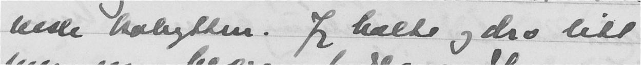lille kahytten. Jeg halte og dro litt
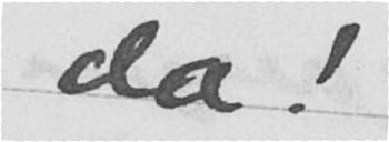da!
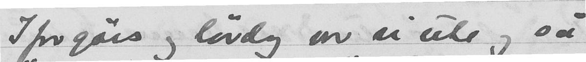Iforgårs og lørdag var vi ute og så
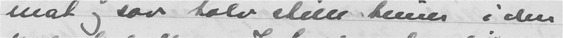mat og sov tolv stille timer i den
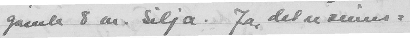gamle 8 m. Silja. Ja, det er sinns-
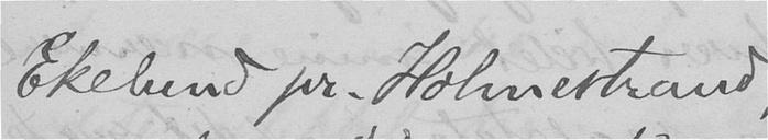Ekelund pr. Holmestrand,
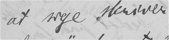at sige skriver
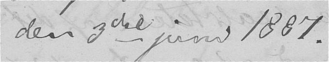den 3die juni 1887.
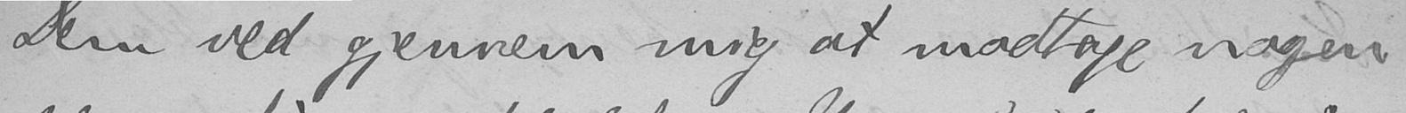Dem ved gjennem mig at modtage nogen
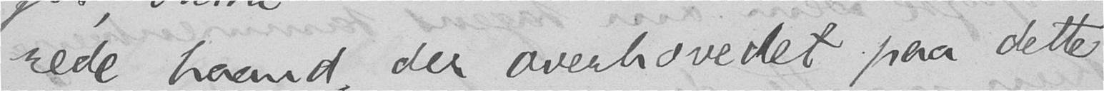rede haand, der overhovedet paa dette
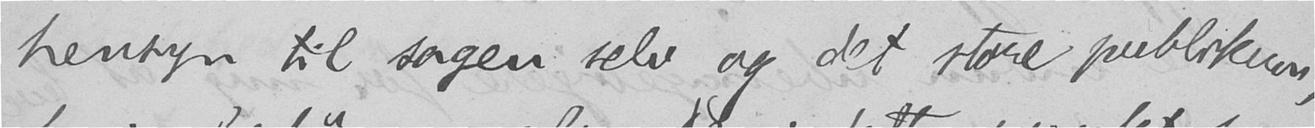hensyn til sagen selv og det store publikum,
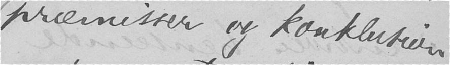præmisser og konklusion
